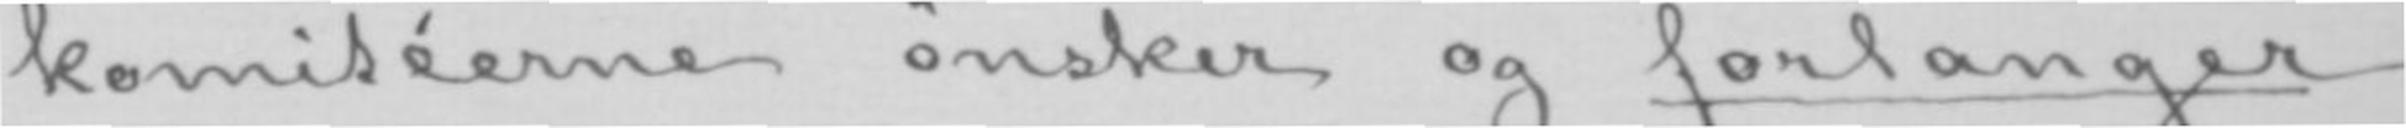komitéerne ønsker og forlanger
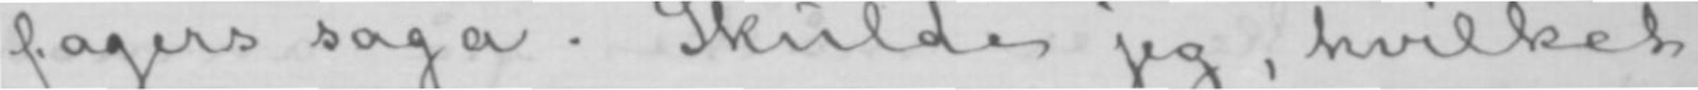fagers saga. Skulde jeg, hvilket
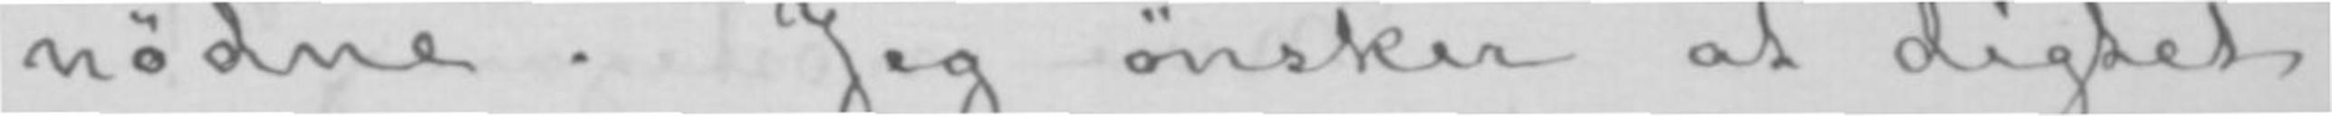nødne. Jeg ønsker at digtet
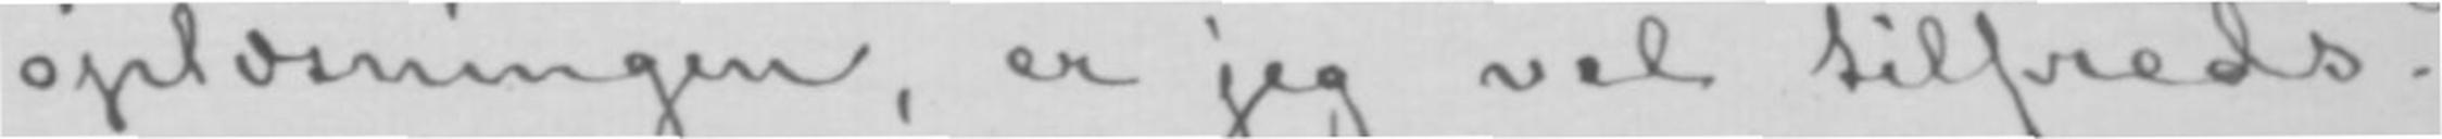oplæsningen, er jeg vel tilfreds.
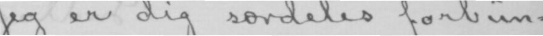Jeg er dig særdeles forbun-
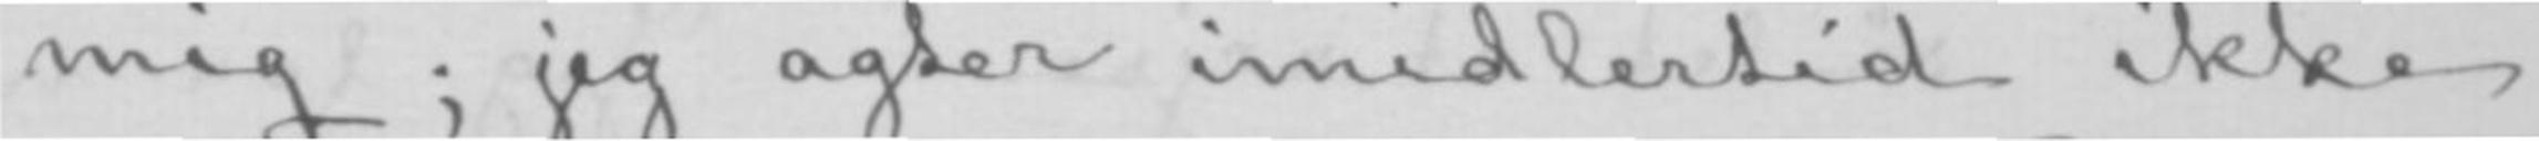mig; jeg agter imidlertid ikke
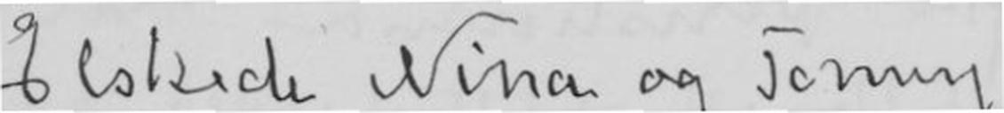Elskede Nina og Tonny!
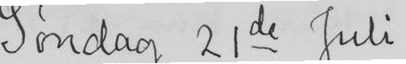Søndag 21de Juli
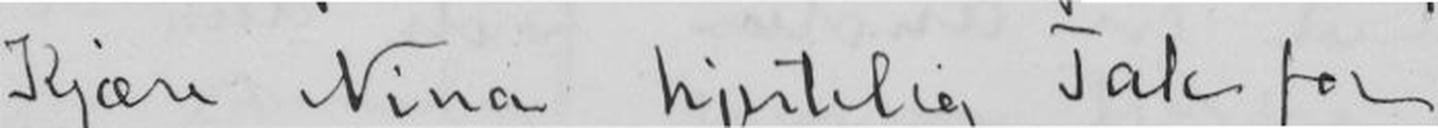Kjære Nina hjertelig Tak for
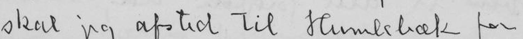skal jeg afsted til Humlebæk for
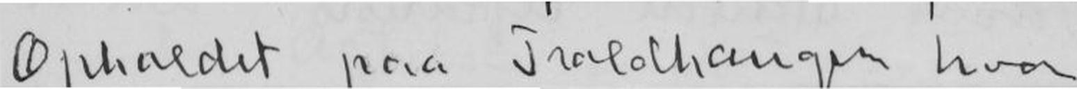Ophaldet paa Troldhaugen hvor
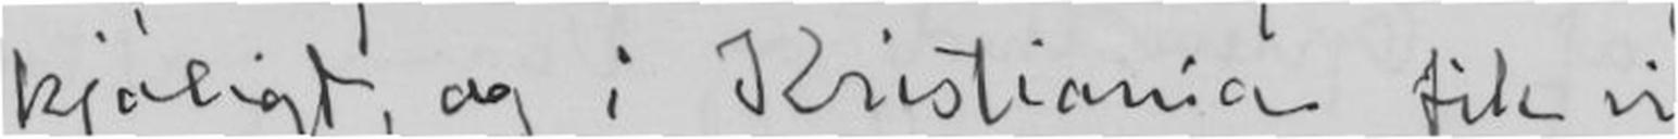kjøligt, og i Kristiania fik vi
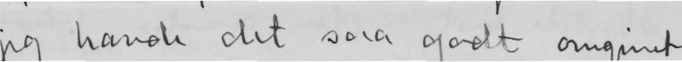jeg havde det saa godt omgivet
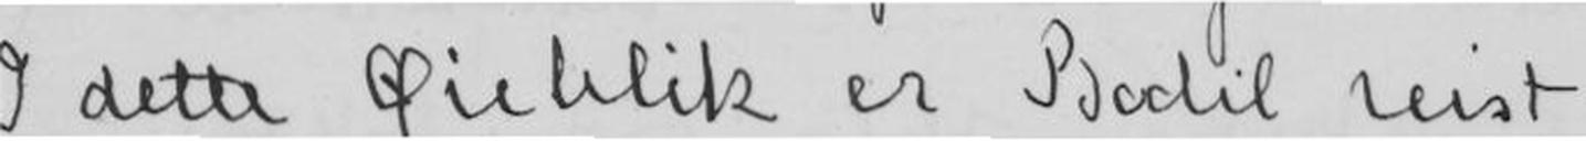Idette Øieblik er Bodil reist
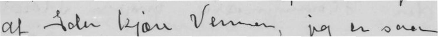af Solen kjære Venner, jeg er saa
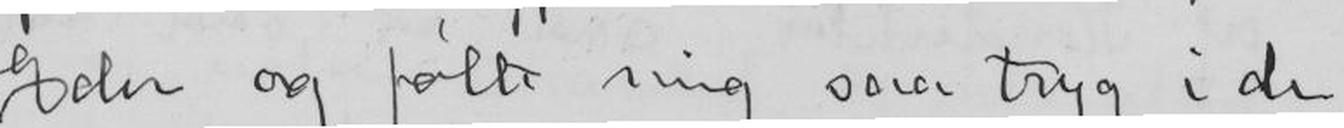Eder og følte mig saa tryg i de
